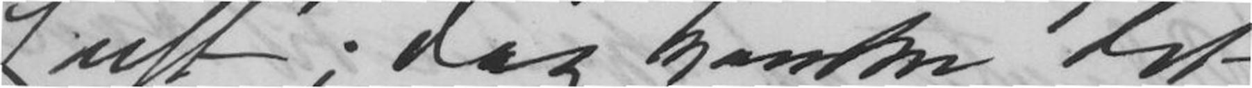Luft; dog kanske det
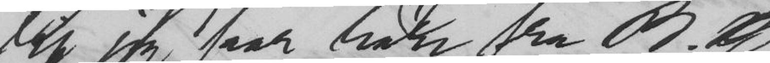til jeg faar høre fra B. B.
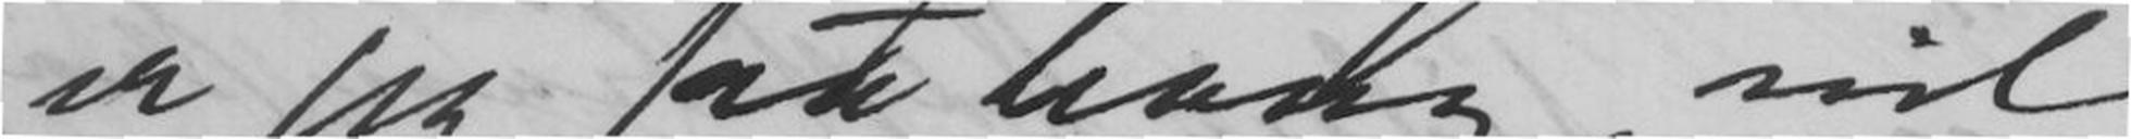er jeg saa bang vil
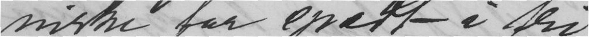virke for spædt i fri
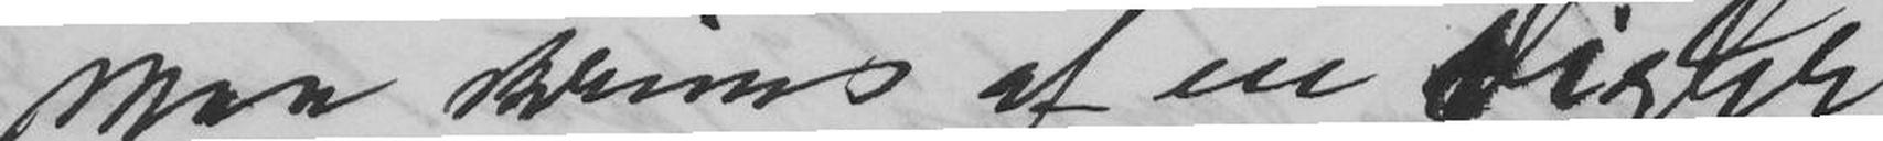maa skrives af en Digter
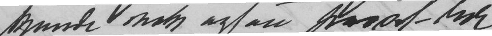kunde nok ogsaa passet her.
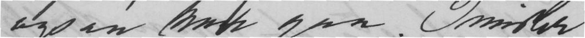ogsaa kan gaa. Imidler-
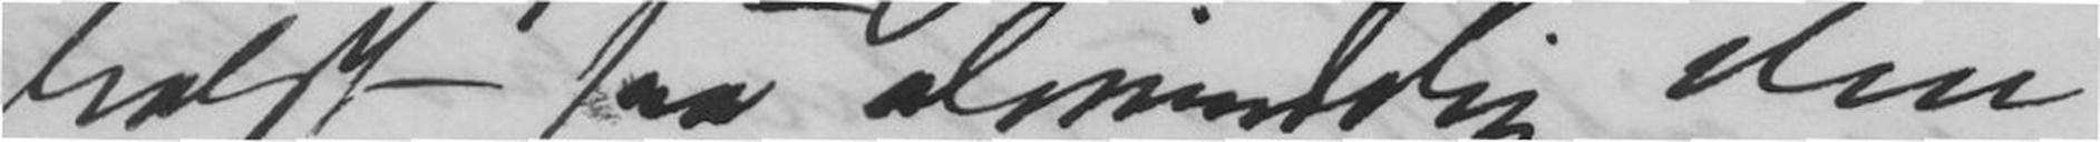holdt saa almindelig den
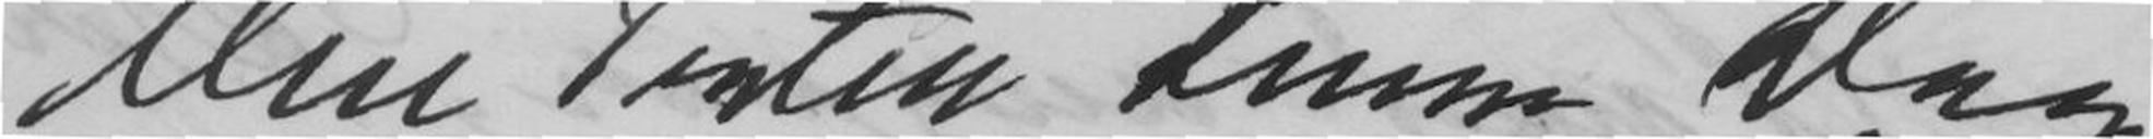Men Texten denne Dag
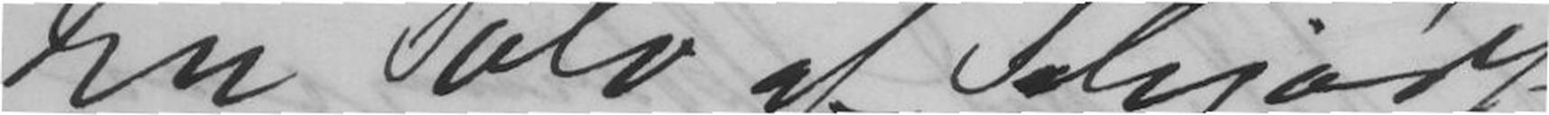En Solo af Schjødt
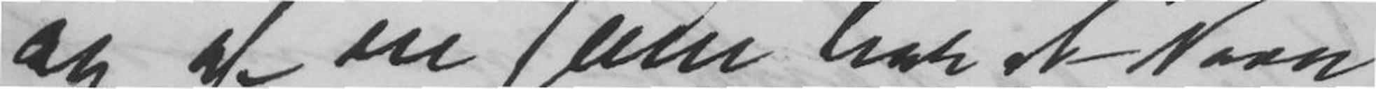og af en som har et Navn.
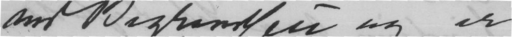ved Begravelsen og er
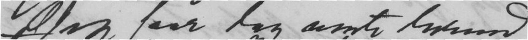Jeg faar dog vente herved
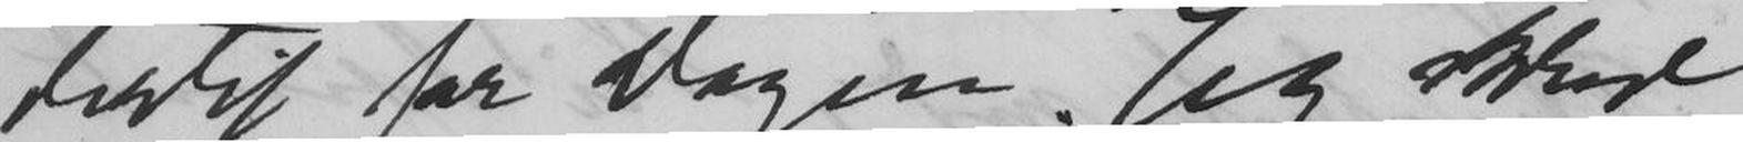dertil for Dagen. Jeg skrev
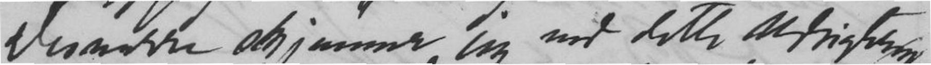Desværre skjønner jeg ved dette Udsigterne
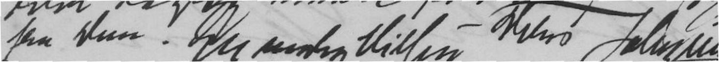fra Dem. En venlig Hilsen Deres John Lund
